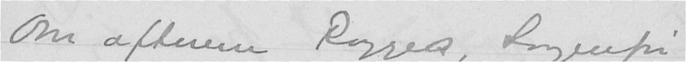Om aftenen Rogges, Sorgenfri
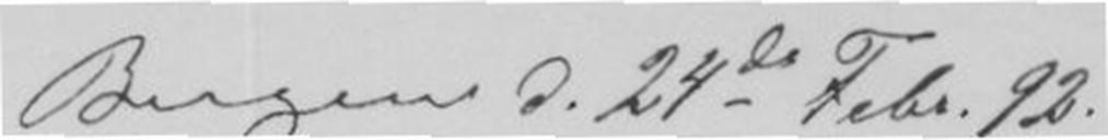Bergen d.24de Febr.92
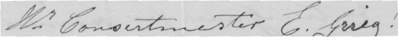Hr. Consertmester E.Grieg!
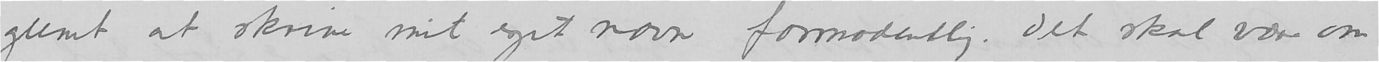glemt at skrive mit eget navn formodentlig. Det skal være om
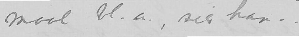maal bl.a., sier han –.
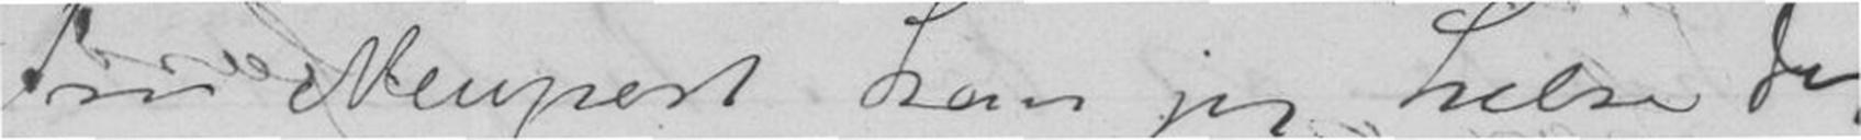Fra Neupert kan jeg hilse dig
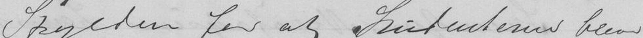Skylden for at Studenterne bleve
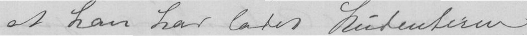at han har ladet Studenterne
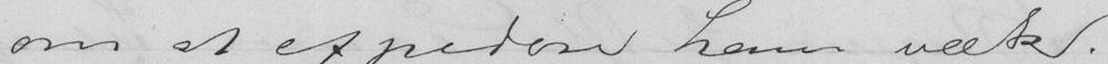om at expedere ham væk.
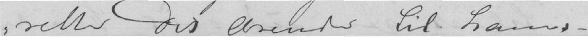rette Dit Ærende til ham. -
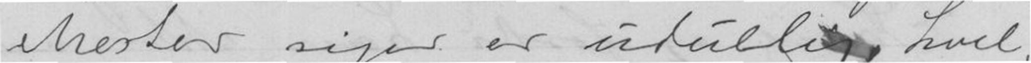Mester siger er uduelig hvil-
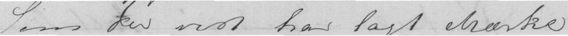Som Du vist har lagt Mærke
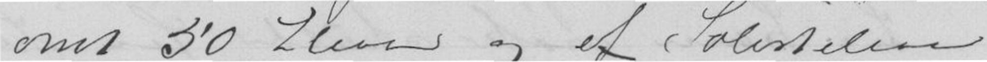omt 50 Elever og af Solisteleve
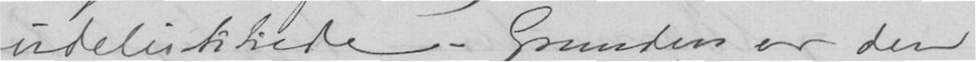udelukkede. Grunden er den
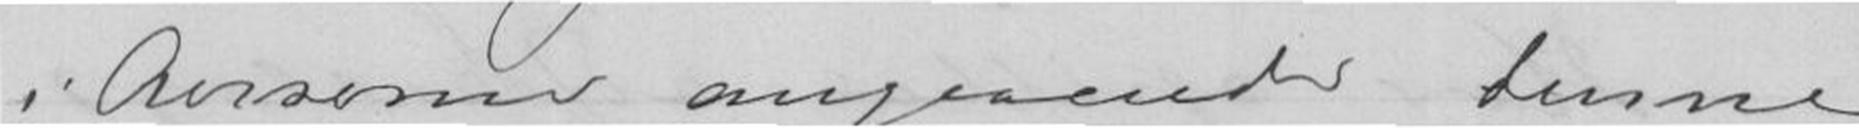i Aviserne angaaende denne
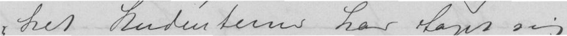ket Studenterne har taget sig
